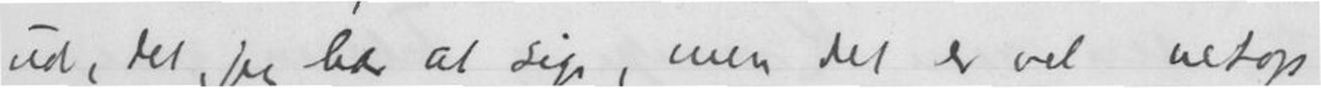ud, det jeg har at sige, men det er vel netop
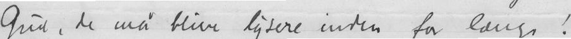Gud, de må blive lysere inden for Længe!
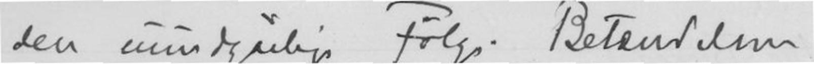den uundgåelige Følge. Betændelsen
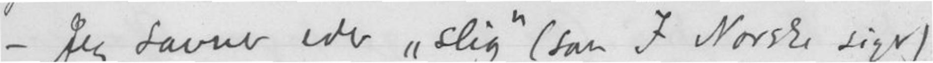-Jeg savner eder slig (saa I Norske siger)
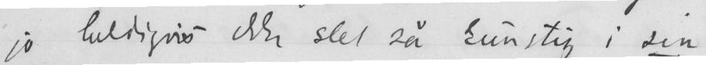jo heldigvis ikke slet så kunstig i sin
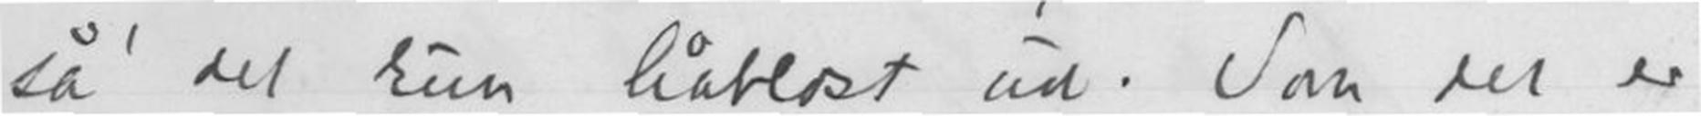så det kun håbløst ud. Som det er
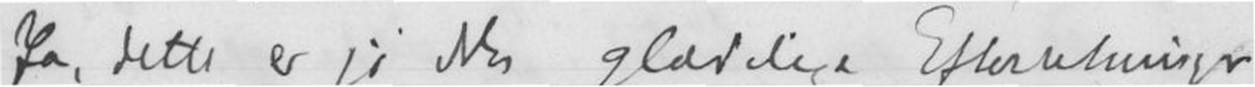Ja, dette er jo ikke glædelige Efterretninger
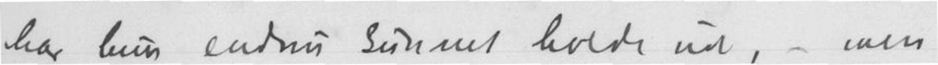har hun endnu kunnet holde ud, men
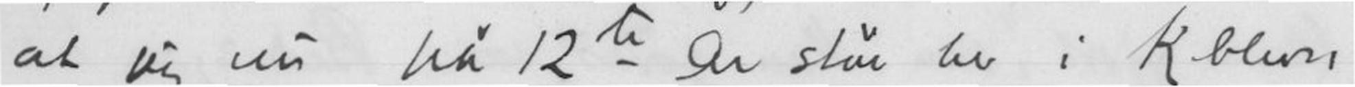at jeg nu på 12te År står her i Kbhvn.
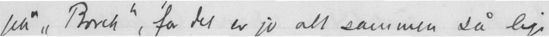på Borch, for det er jo alt sammen så lige
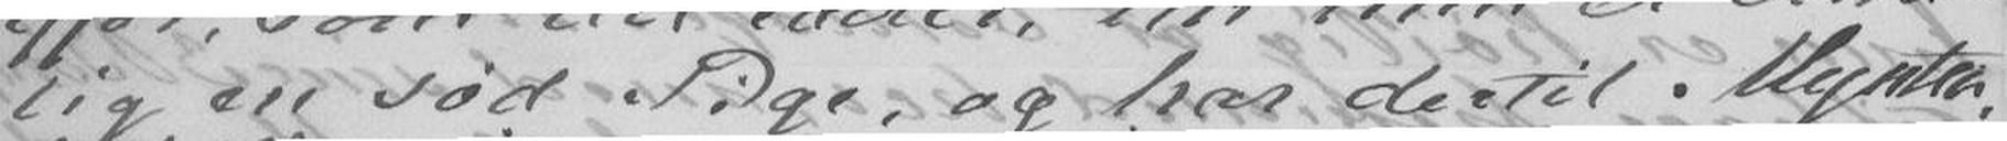lig en sød Pige, og har dertil Mynten,
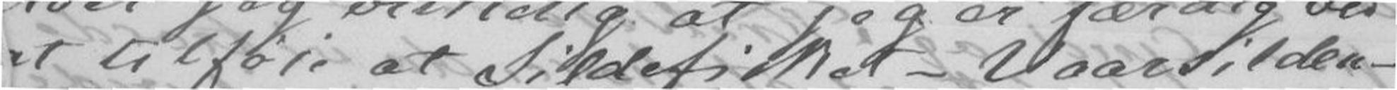at tilføie at sildefisket - Vaarsilden -
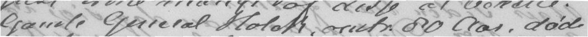Gamle General Holck, omtr. 80 Aar, døde
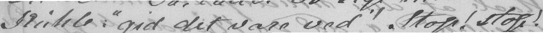Kühle: "gid det vare ved"! Stop! stop!
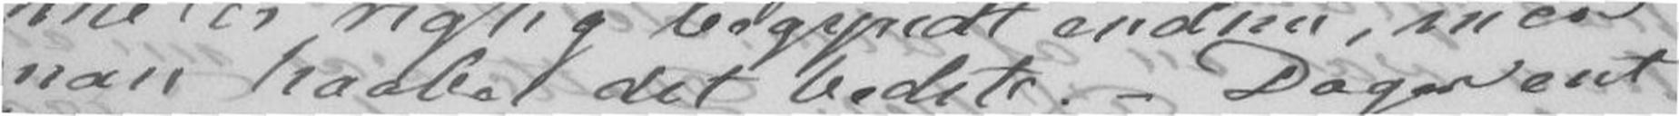man haabe det bedste. - Dog vent
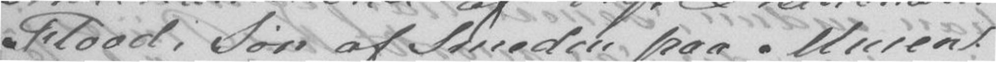Flood, Søn af Smeden paa Muren
